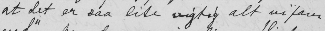at det er saa lite vigtig alt vi farer
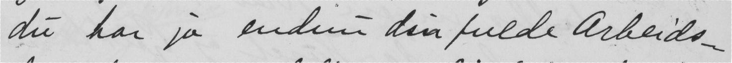du har jo endnu din fulde Arbeids-
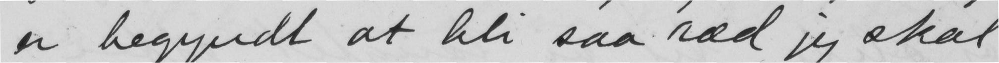er begyndt at bli saa ræd jeg skal
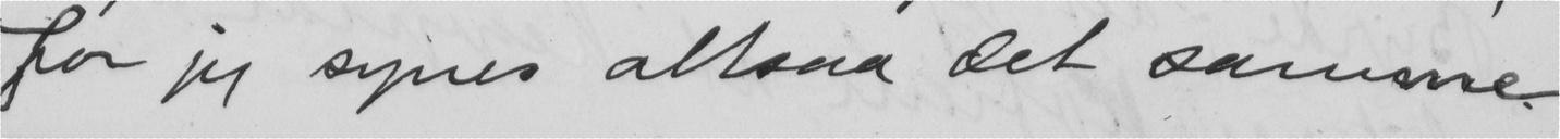for jeg synes altsaa det samme.
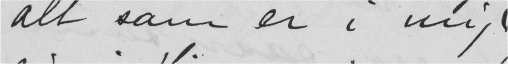alt som er i mig
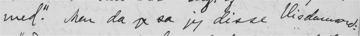med." Men da  sa jeg disse Visdomord:
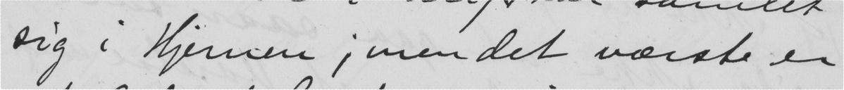sig i Hjernen; men det værste er
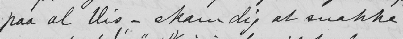paa al Vis - skam dig at snakke
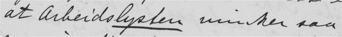at Arbeidslysten minker saa
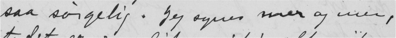saa sørgelig. Jeg synes mer og mer,
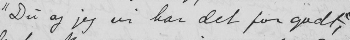"Du og jeg vi har det for godt;"
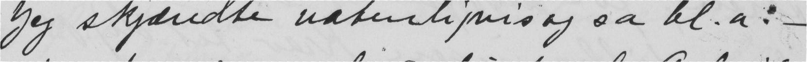Jeg skjændte naturligvis og sa bl.a:-
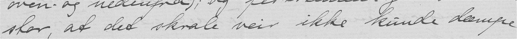stor, at det skrale veir ikke kunde dæmpe
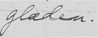glæden.
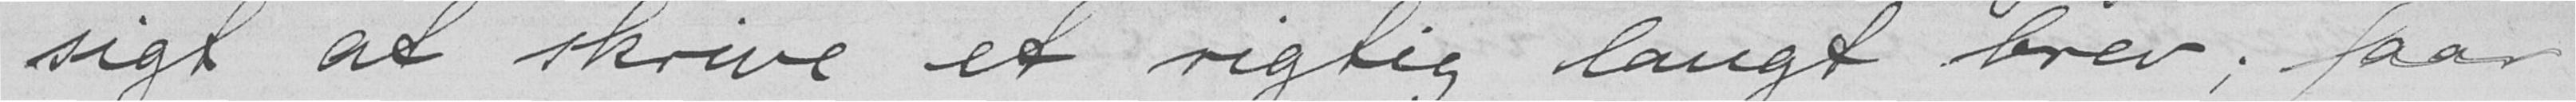sigt at skrive et rigtig langt brev; faar
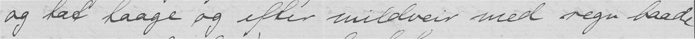og tæt taage og efter mildveir med regn baade
